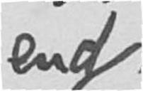end
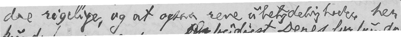dre rigelige, og at ogsaa rene ubetydeligheder her
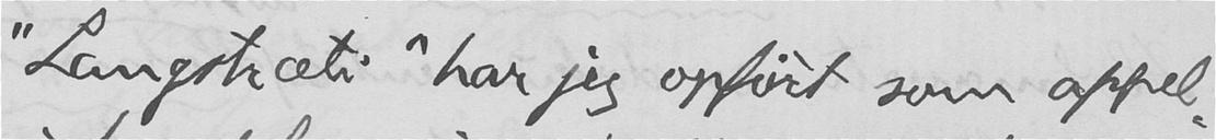"Langstræti" har jeg opført som appel-
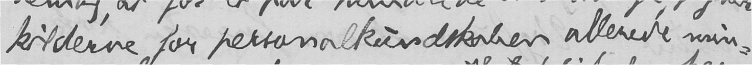kilderne for personalkundskaben allerede min-
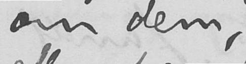om dem,
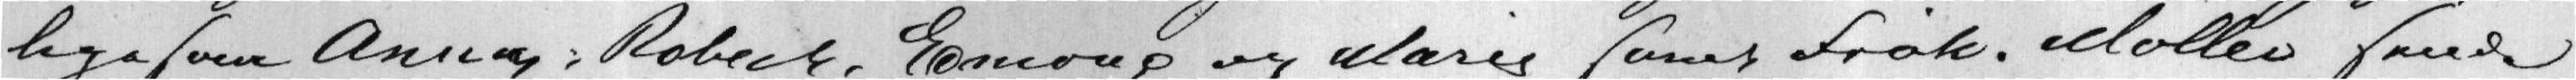ligesom Anna, Robert, Edmund og Marie samt Frøk. Möller sende
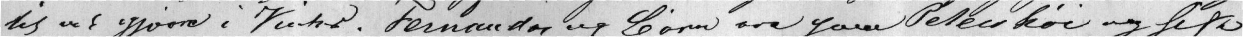til at gjøre i Vinter. Fernanda og Børn ere paa Petershøi og hilst
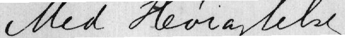Med Høiagtelse
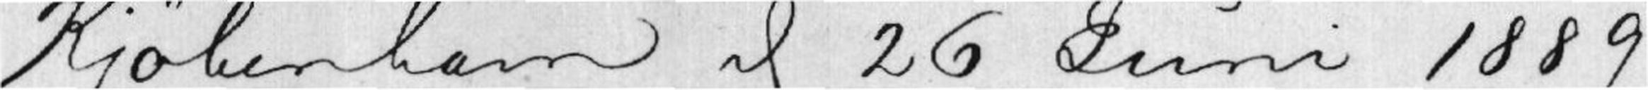Kjøbenhavn d 26 Juni 1889
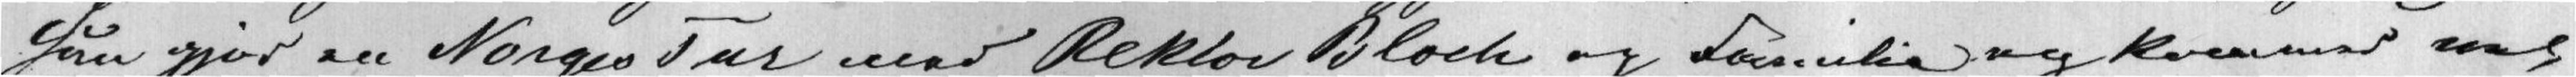Hun gjør en Norges Tur med Rektor Block og Familie og kommer vel
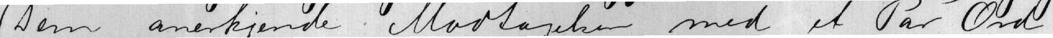Dem anerkjende Modtagelsen med et Par Ord.
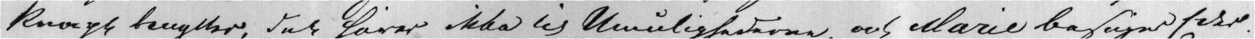knapt benytter, det hører ikke til Umulighederne, at Marie besøger Eder.
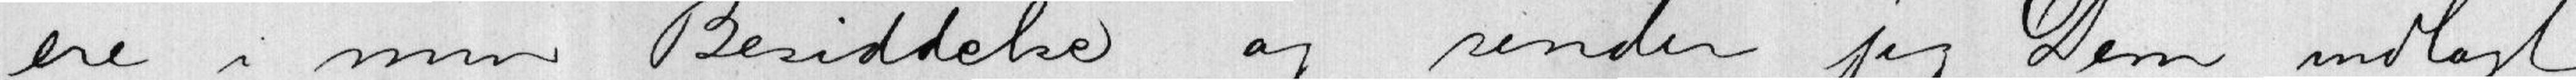ere i min Besiddelse og sender jeg Dem indlagt
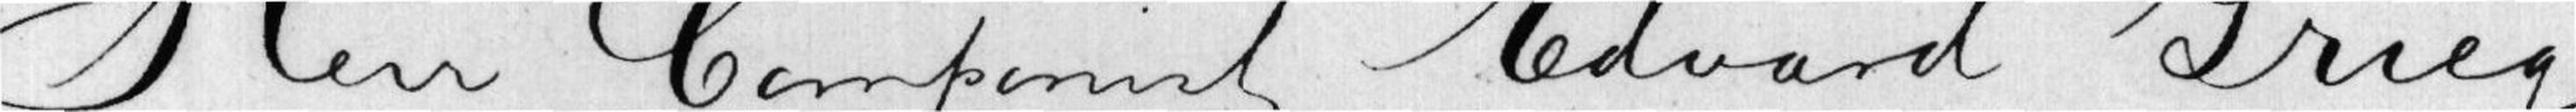Henriques KjøbenhavnHerr Componist Edvard Grieg.
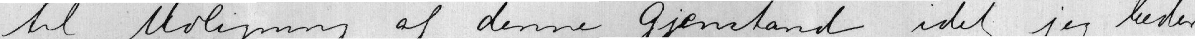til Udgangen af denne Gjenstand idet jeg beder
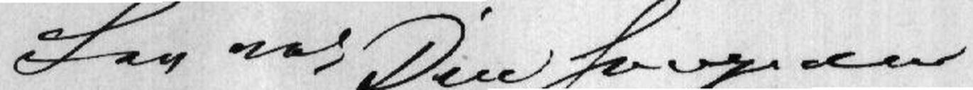Lev vel Din hengivne
